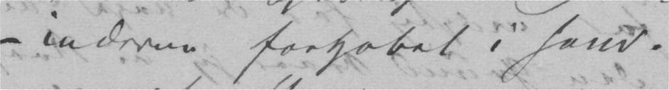-inderne forgabet i ham.
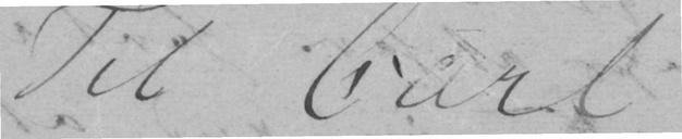Til Carl
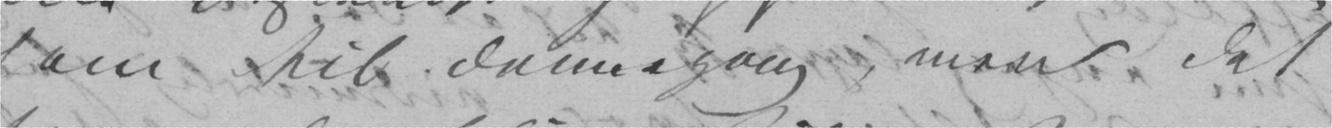ham til dennegang, men det
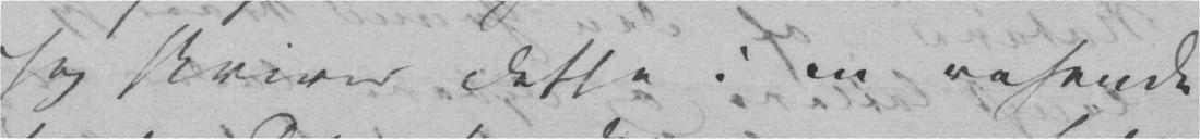Jeg skriver dette i en rasende
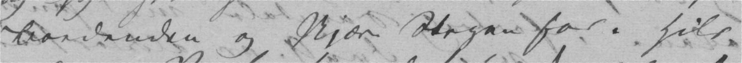Bordenden og skjære Stegen for. Hils
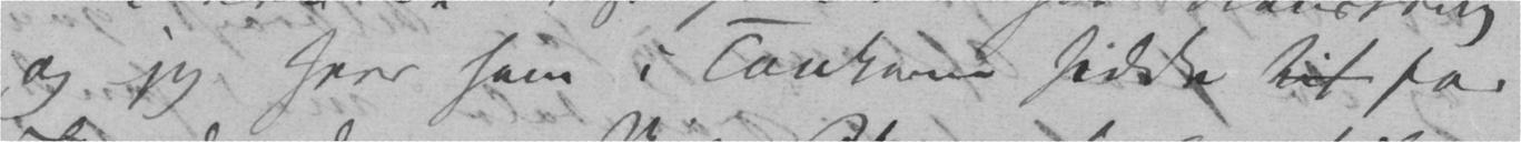og jeg seer ham i Tankerne sidde til for
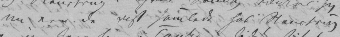nu ere de vist samlede hos Stenstrup
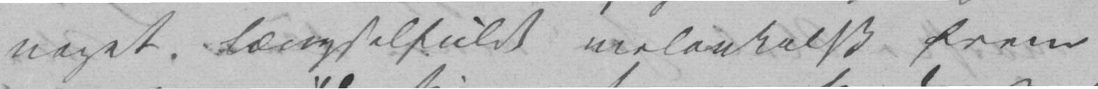noget længselfuldt melankolsk frem
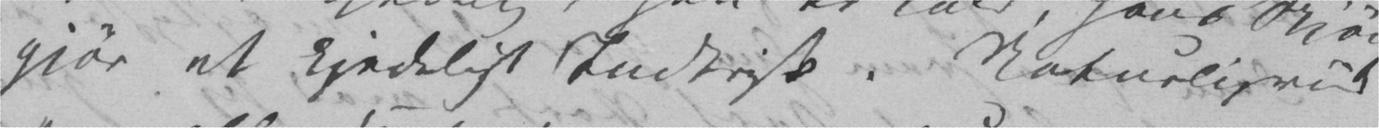gjør et kjedeligt Indtryk. Naturligviis
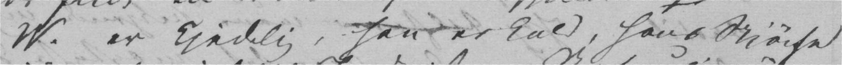W. er kjedelig, han er kold, hans Skjønhed
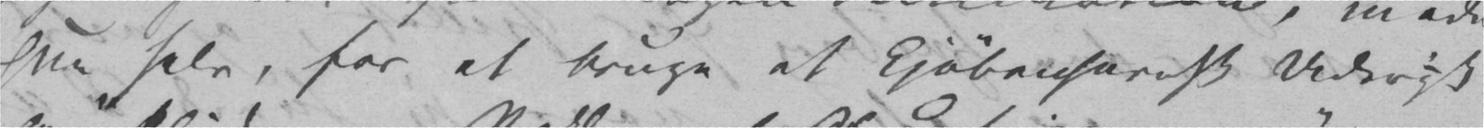hun selv, for at bruge et kjøbenhavnsk Udtryk
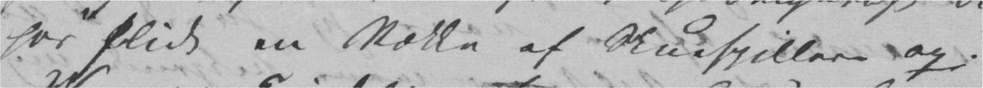har «slidt en Række af Skuespillere op.»
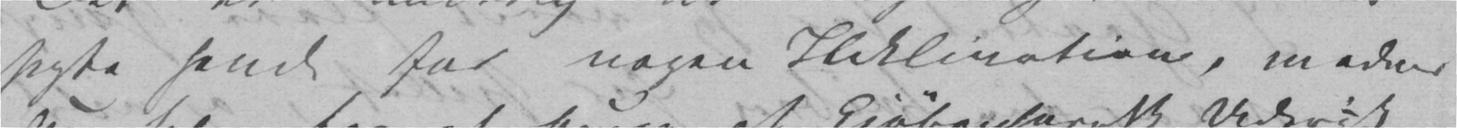sigte hende for nogen Inklination, medens
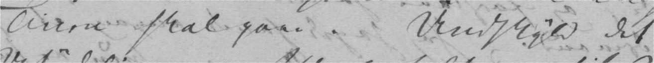Time skal gaae. Undskyld det
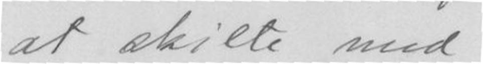at skilte med.
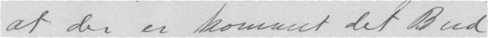at der er kommet det Bud
